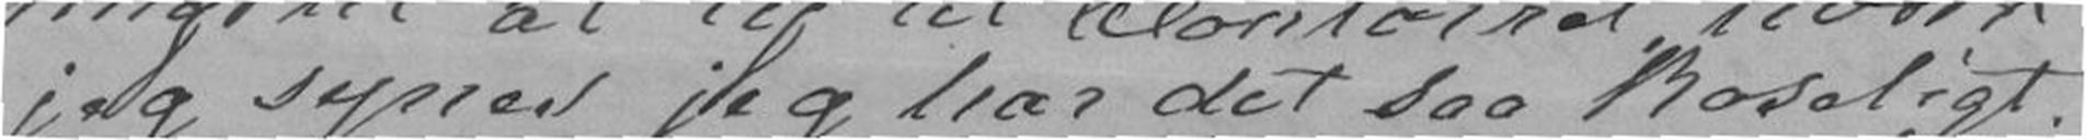jeg synes jeg har det saa koseligt.
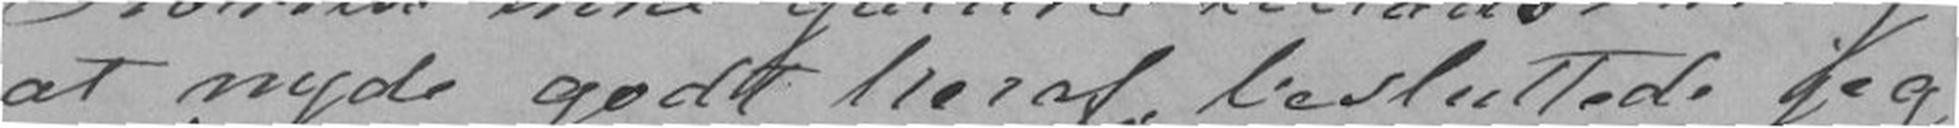at nyde godt heraf besluttede jeg
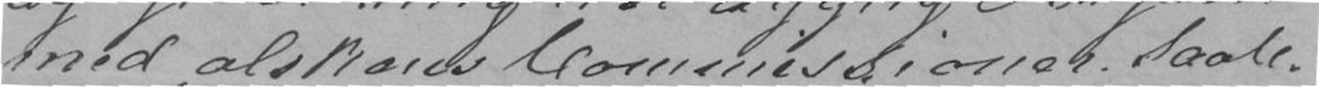med alskens Commissioner. Saale-
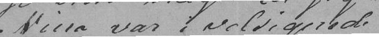Nina var velsignede
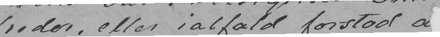heder, eller ialfald forstod a
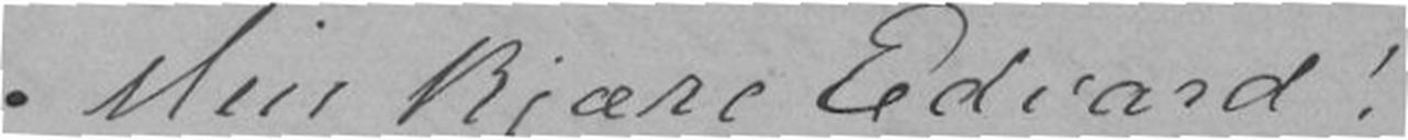Min kjære Edvard!
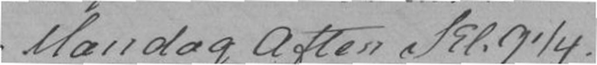Mandag Aften Kl.9¼.
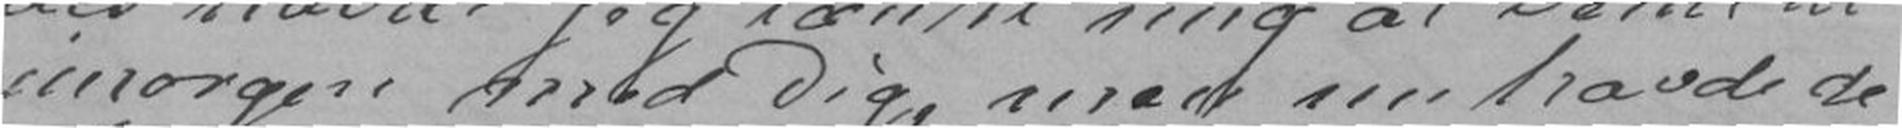imorgen med Dig, men nu havde de
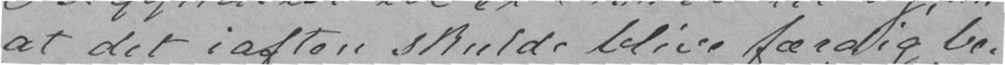at det iaften skulde blive færdig be-
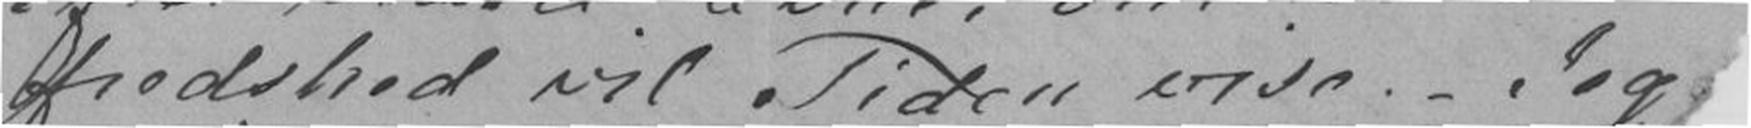fredshed vil Tiden vise. - Jeg
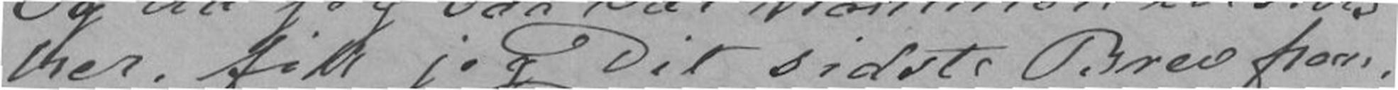her, fik jeg Dit sidste Brev frem-
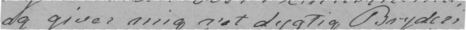og giver mig ret dygtig Bryderi
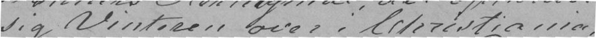sig Vinteren over i Christiania,
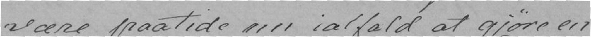være paatide nu ialfald at gjøre en
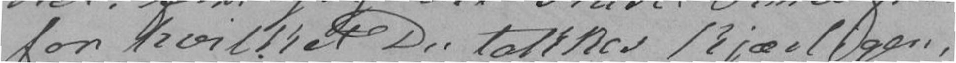for hvilket Du takker kjærligen,
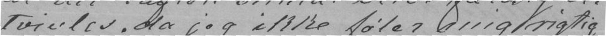tviles, da jeg ikke føler mig rigtig
